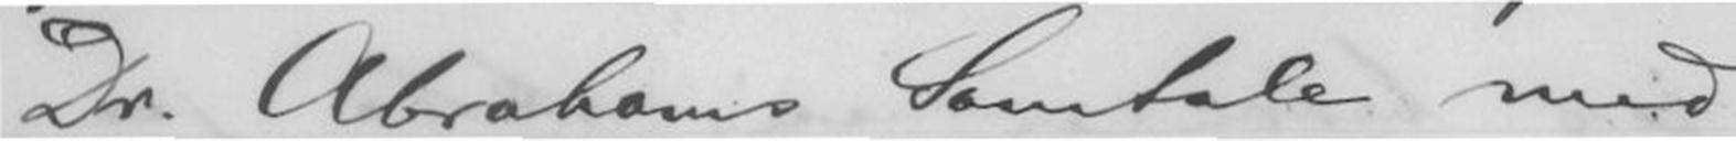Dr. Abrahams Samtale med
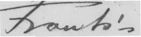Frants's.
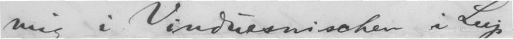mig i Vinduesnischen i Leip-
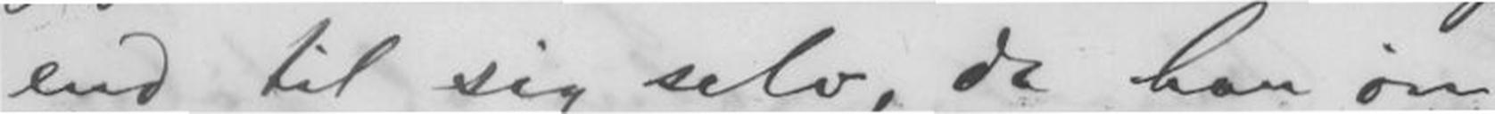end til sig selv, da han øn-
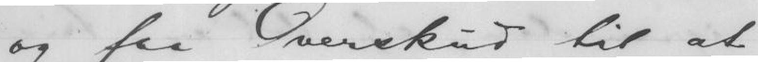og faa Overskud til at
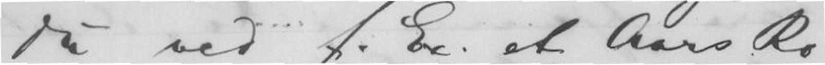Du ved f.Ex. et Aars Ro
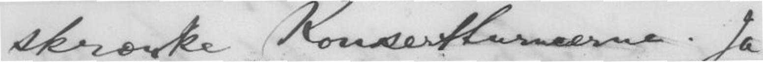skrænke Konsertturneerne. Ja
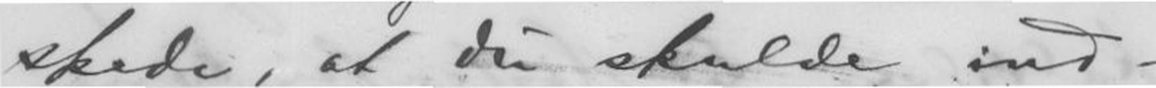skede, at du skulde ind-
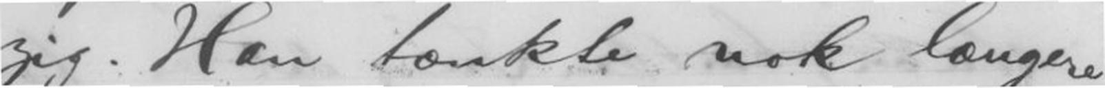zig. Han tænkte nok længere
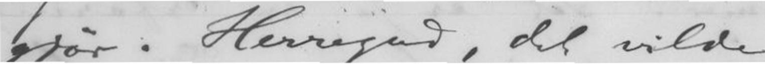gjør. Herregud, det vilde
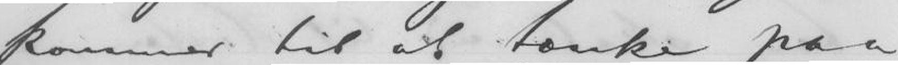kommer til at tænke paa
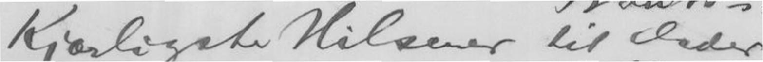Kjærligste Hilsener til Eder
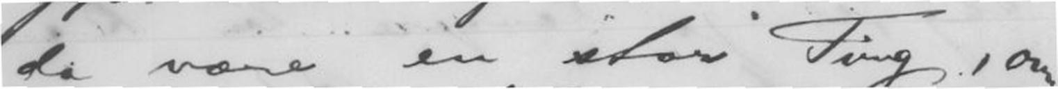da være en stor Ting, om
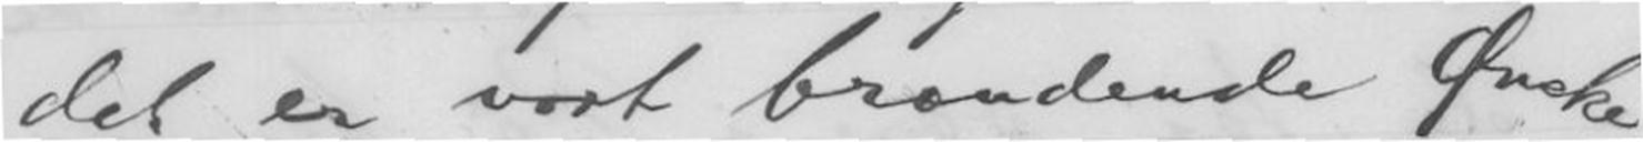det er vort brændende Ønske,
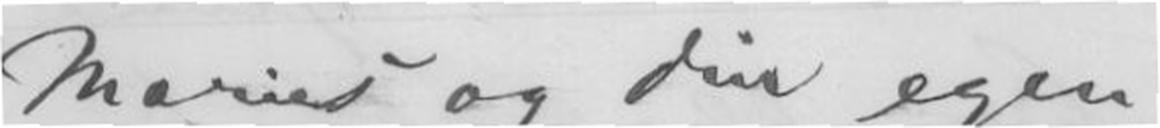Maries og din egen
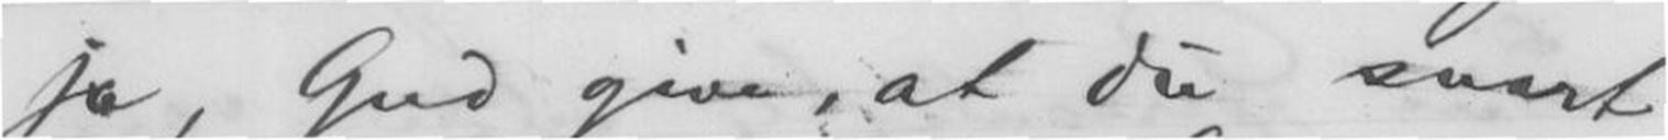ja, Gud give, at du snart
